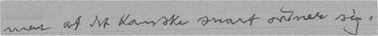men at det kanske snart ordner sig.
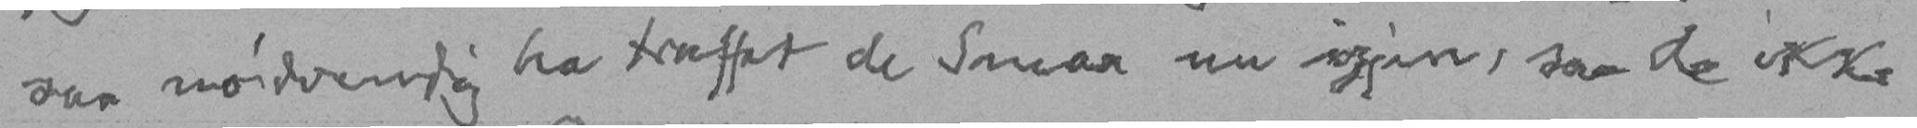saa nødvendig ha truffet de Smaa nu igjen, saa de ikke
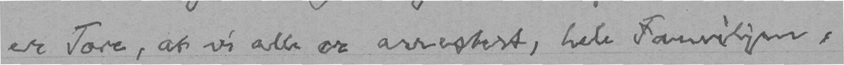er Tore, at vi alle er arrestert, hele Familjen,
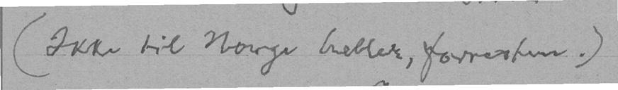(Ikke til Norge heller, forresten.)
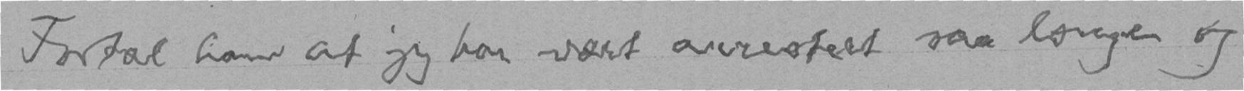Fortæl ham at jeg har vært arrestert saa længe og
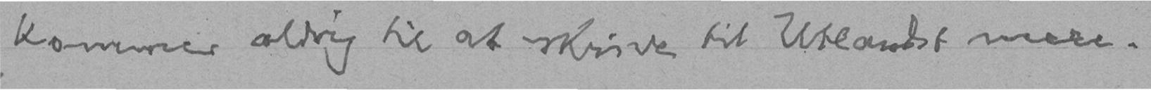kommer aldrig til at skrive til Utlandet mere.
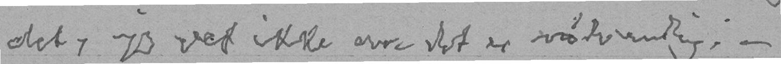det, jeg vet ikke om det er nødvendig; -
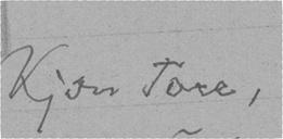Kjære Tore,
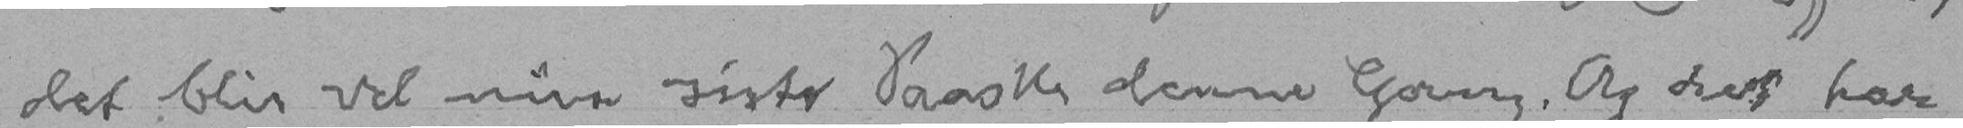det blir vel min siste Paaske denne Gang. Og det har
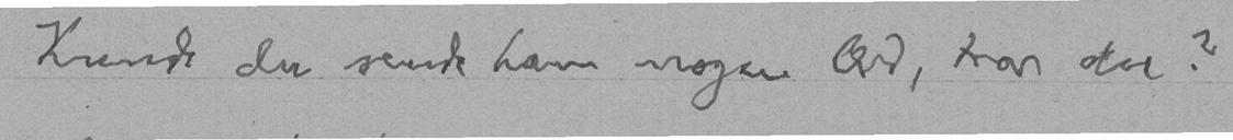Kunde du sende ham nogen Ord, tror du?
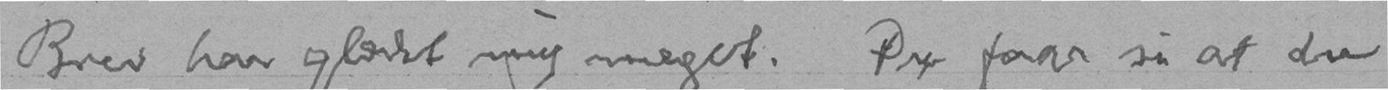Brev har glædet mig meget. Du faar si at du
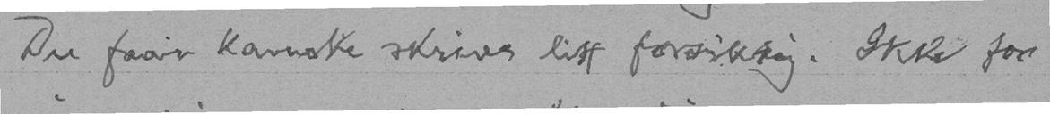Du faar kanske skrive litt forsiktig. Ikke for
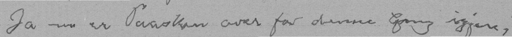Ja nu er Paasken over for denne Gang igjen,
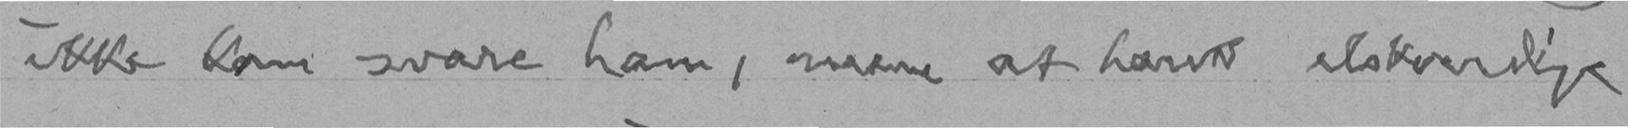ikke kan svare ham, mene at hans elskverdige
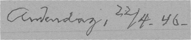Andendag, 22/4. 46.
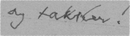og takker!
